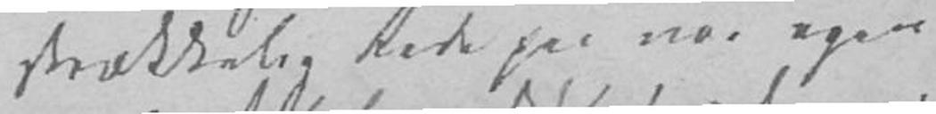strækkelig Rede paa vor egen
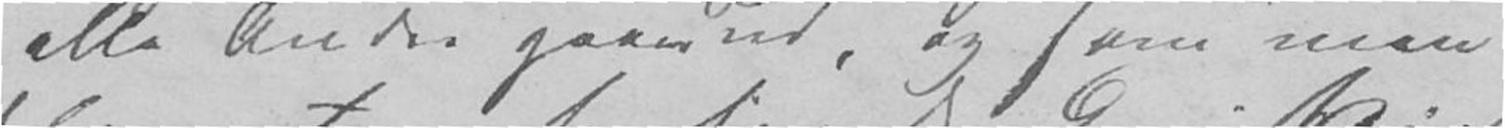alle Andre gaar ud, og som man
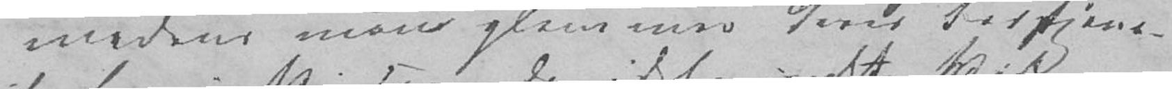medens man glemmer deres Fortjene-
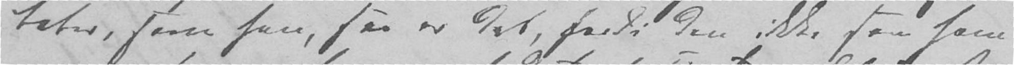tater, som han, saa er det, fordi den ikke som ham
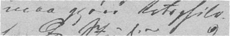maa gjøre Retsphilo-
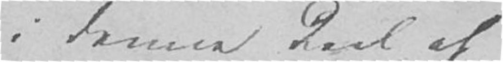i denne Deel af
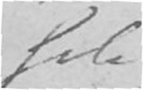hele
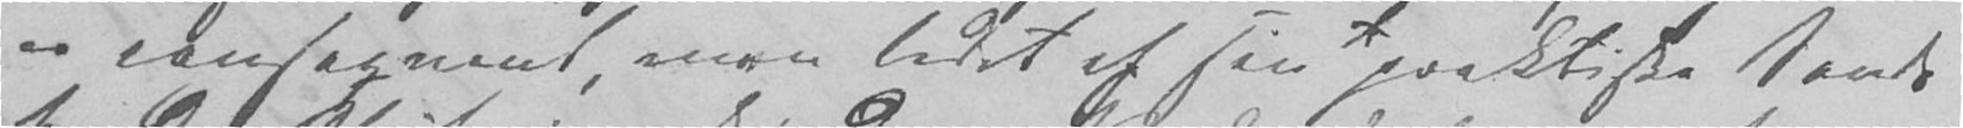er conseqvent, men ledet af sin praktiske Sands
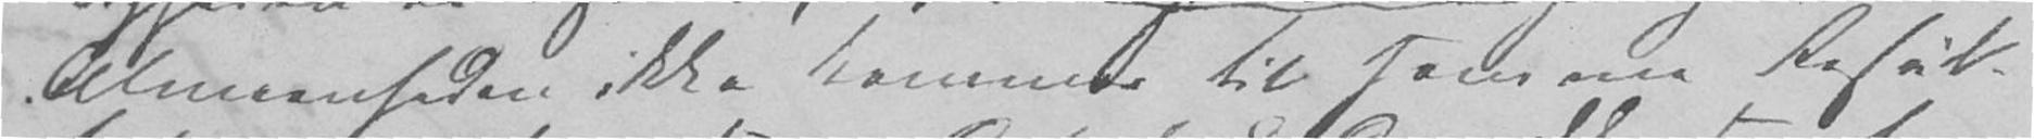Almeenheden ikke kommer til samme Resul-
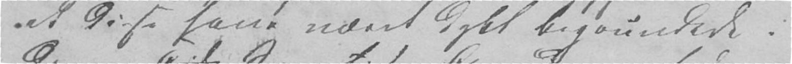at disse have været dybt begrundede i
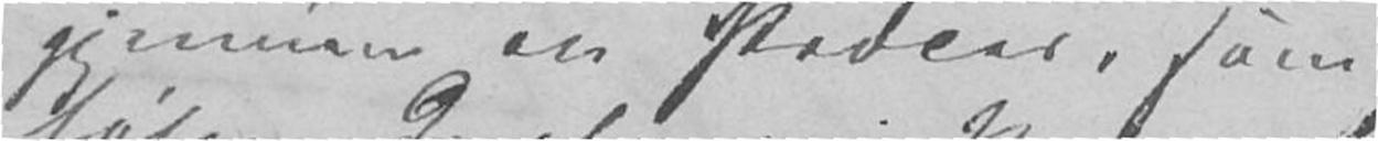gjennem en Proces, som
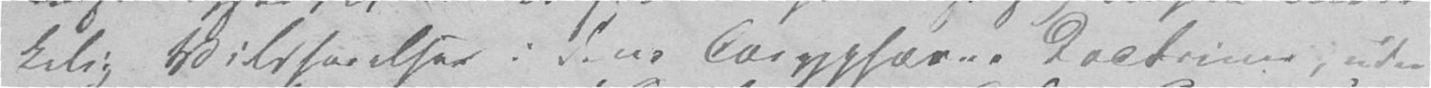kelig Vildfarelser i dens Coryphærne Doctriner, uden
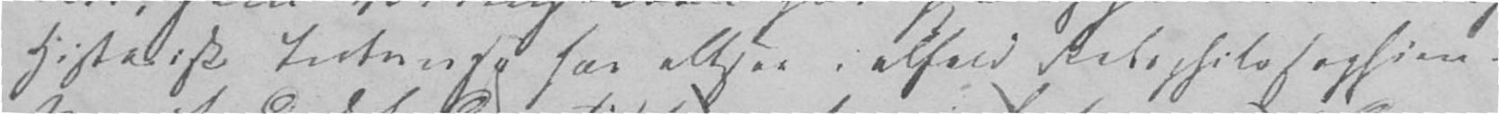Historisk Interesse har altsaa i alfald Retsphilosophien.
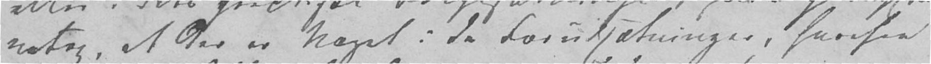netop, et der er Noget i de Forudsætninger, hvorfra
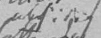alsidig
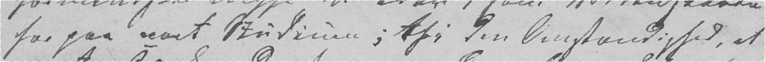har paa vort Studium; thi den Omstændighed, at
